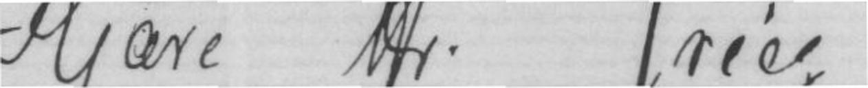Kjære Hr. Grieg
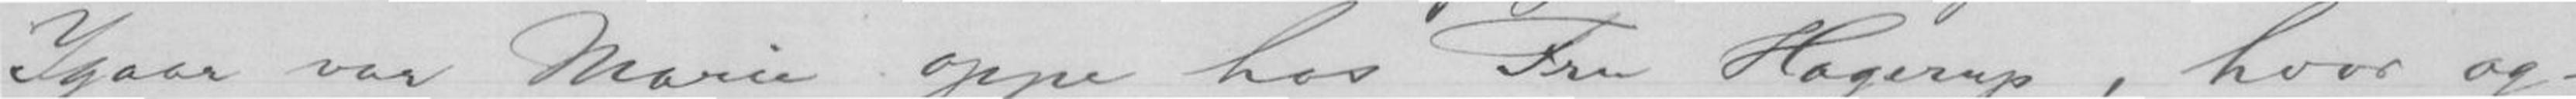Igaar var Marie oppe hos Fru Hagerup, hvor og-
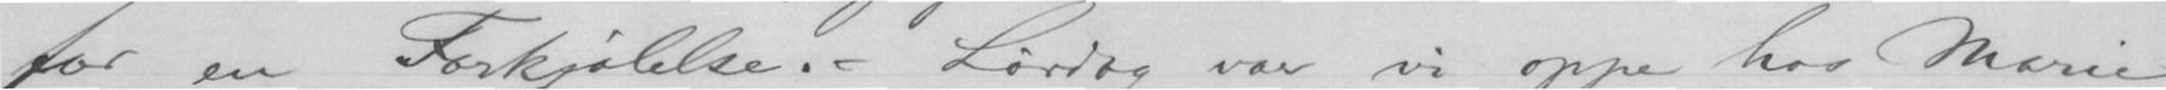for en Forkjølelse. - Lørdag var vi oppe hos Marie
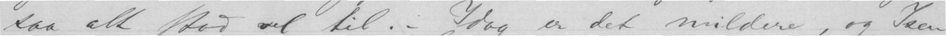saa alt stod vel til. - Idag er det mildere, og Isen
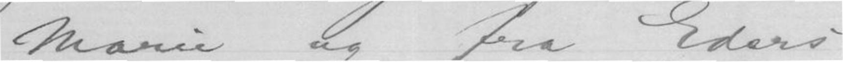Marie og fra Eders
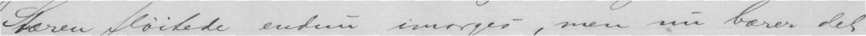Stæren fløitede endnu imorges, men nu bærer det
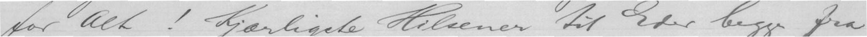for Alt! Kjærligste Hilsener til Eder begge fra
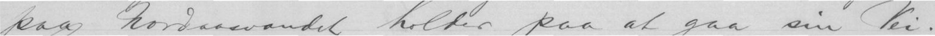paa Nordaasvandet holder paa at gaa sin Vei.
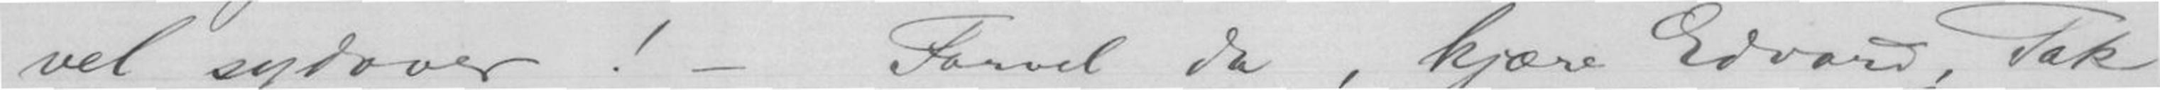vel sydover! - Farvel da, kjære Edvard, Tak
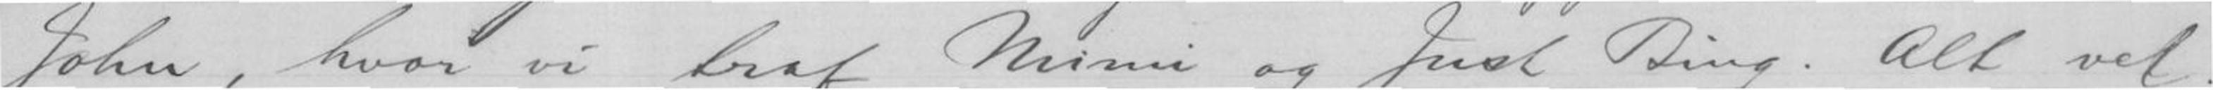John, hvor vi traf Mimi og Just Bing. Alt vel.
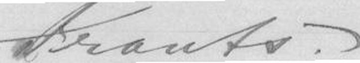Frants.
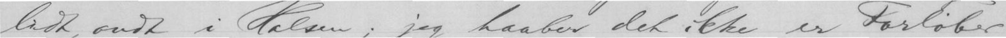lidt ondt i Halsen; jeg haaber det ikke er Forløber
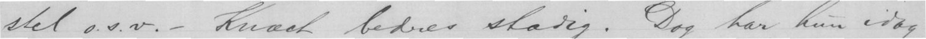stel o.s.v. - Knæet bedres Stadig. Dog har hun idag
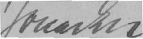Jonas Lie
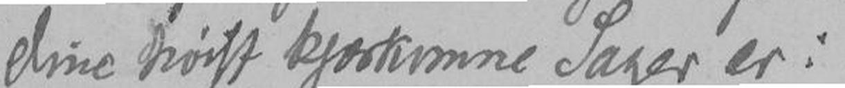dine høist kjærkomne Sager er:
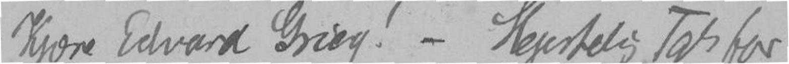Kjære Edvard Grieg ! - Hjertelig Tak for
